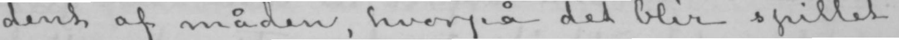dent af måden, hvorpå det blir spillet.
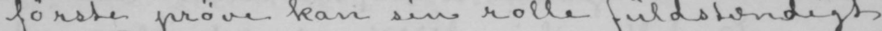første prøve kan sin rolle fuldstændigt.
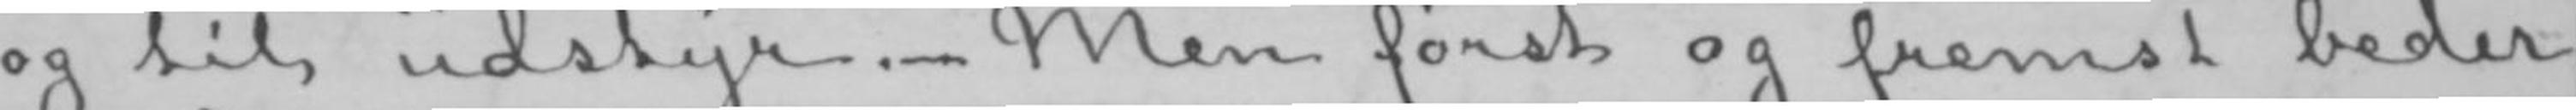og til udstyr.- Men først og fremst beder
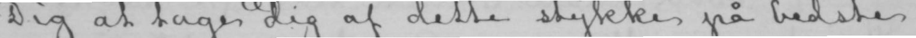Dig at tage dDig af dette stykke på bedste
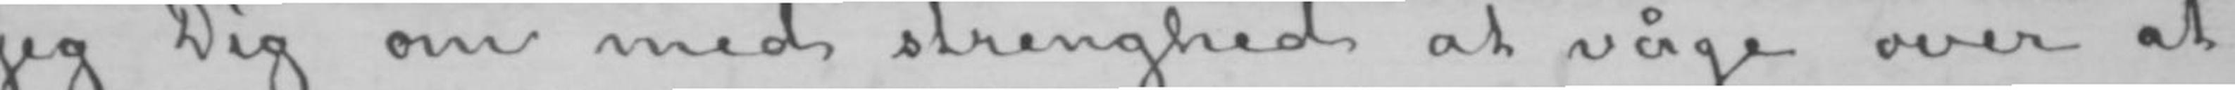jeg Dig om med strenghed at våge over at
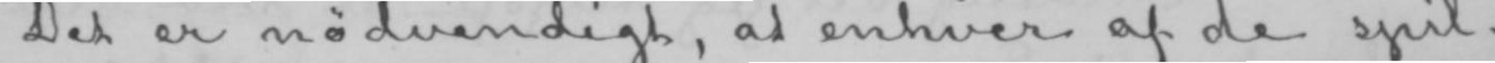Det er nødvendigt, at enhver af de spil-
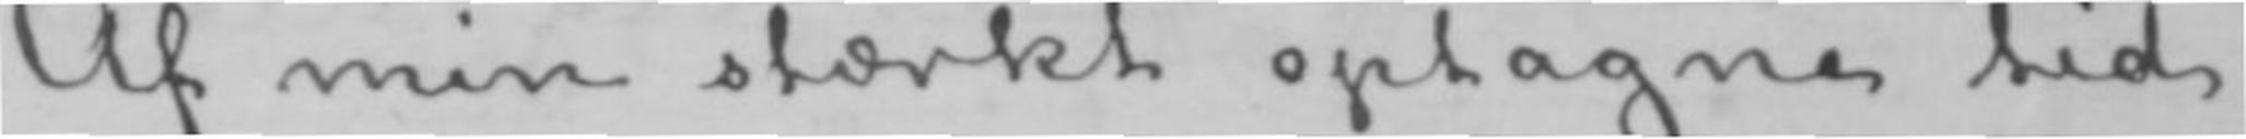Af min stærkt optagne tid
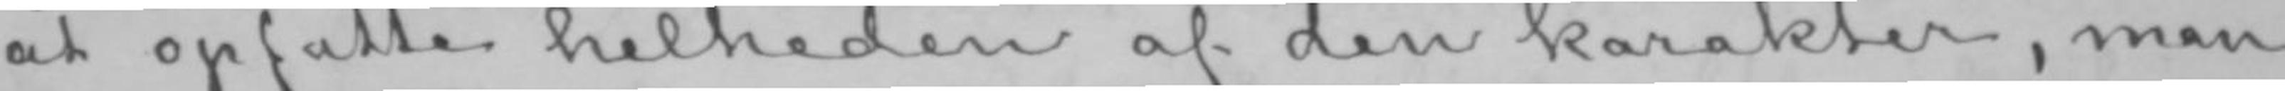at opfatte helheden af den karakter, man
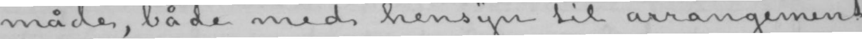måde, både med hensyn til arrangement
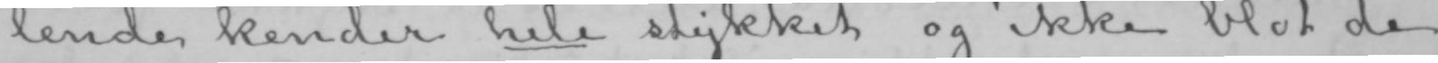lende kender hele stykket og ikke blot de
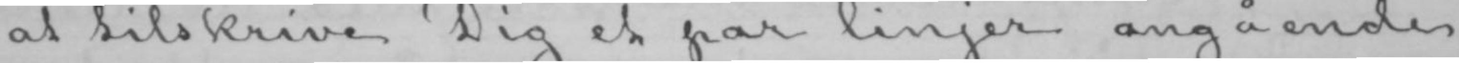at tilskrive Dig et par linjer angående
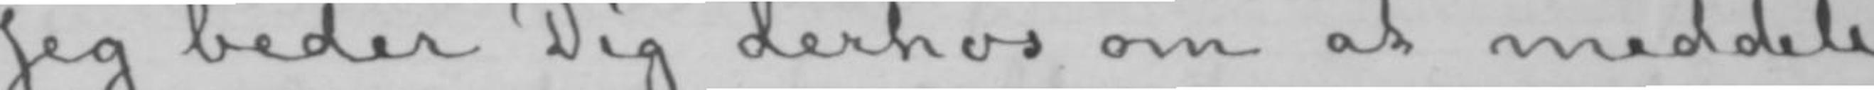Jeg beder Dig derhos om at meddele
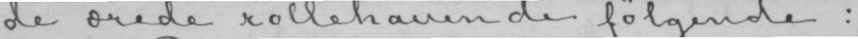de ærede rollehavende følgende:
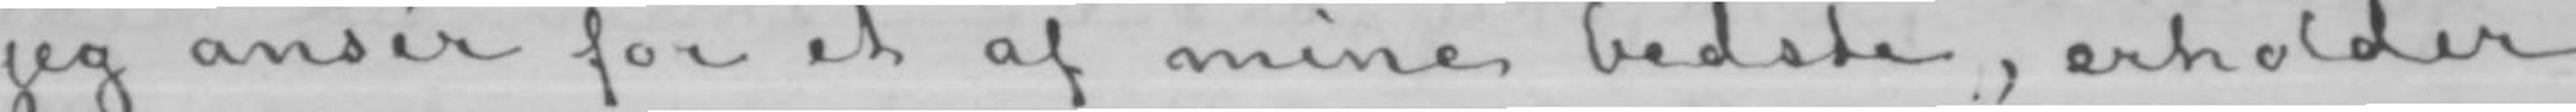jeg ansér for et af mine bedste, erholder
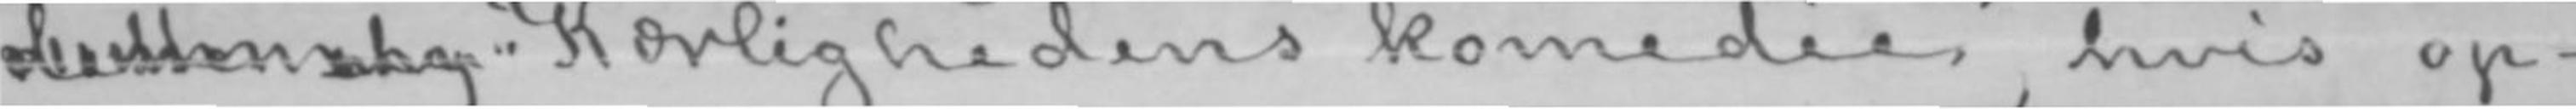dette sty «Kærlighedens komedie», hvis op-
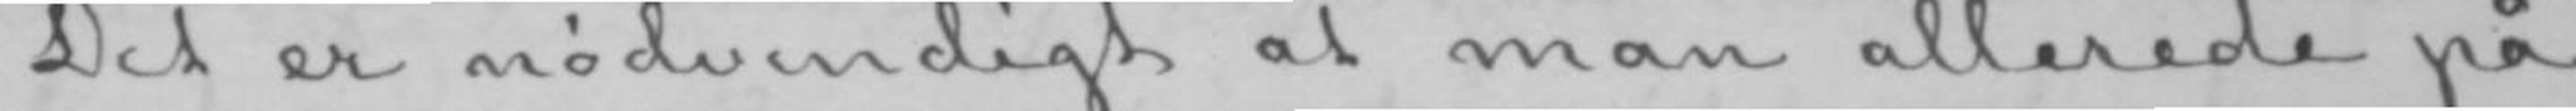Det er nødvendigt at man allerede på
